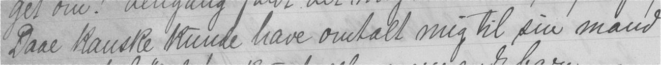Daae kanske kunde have omtalt mig til sin mand
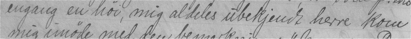engang en høi, mig aldeles ubekjendt herre kom
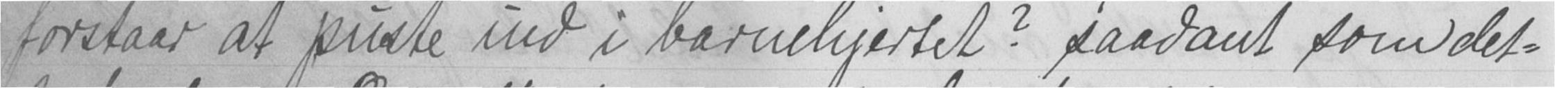forstaar at puste ind i barnehjertet? saadant som det-
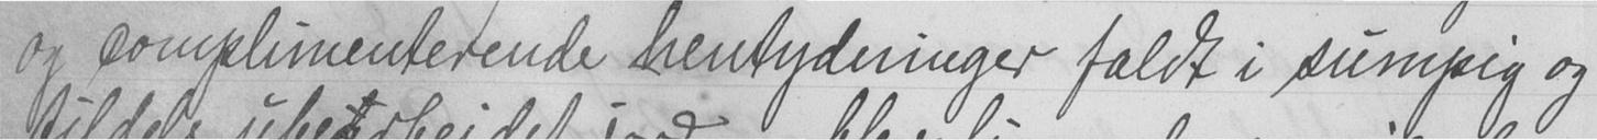og complimenterende hentydninger faldt i sumpig og
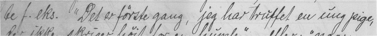te f.eks. "Det er første gang, jeg har truffet en ung pige,
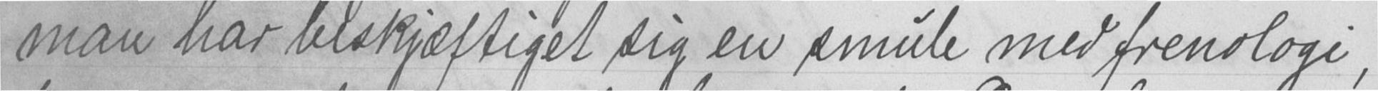man har beskjæftiget sig en smule med frenologi,
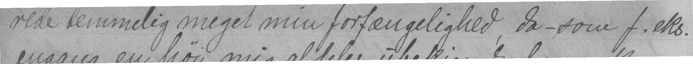rede temmelig meget min forfængelighed, da - som f. eks.
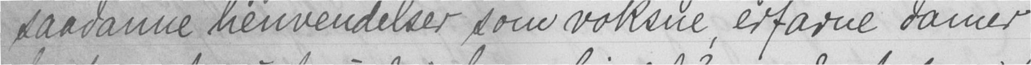saadanne henvendelser som voksne erfarne damer
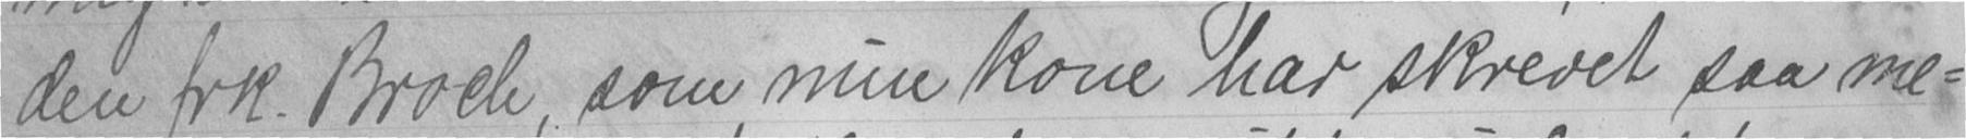den frk. Broch, som min kone har skrevet saa me-
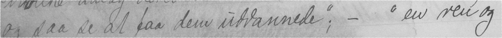og saa se at faa dem uddannede"; - "en ren og
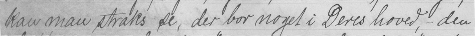kan man straks se, der bor noget i Deres hoved, - den
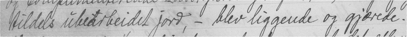tildels ubearbeidet jord, - blev liggende og gjærede.
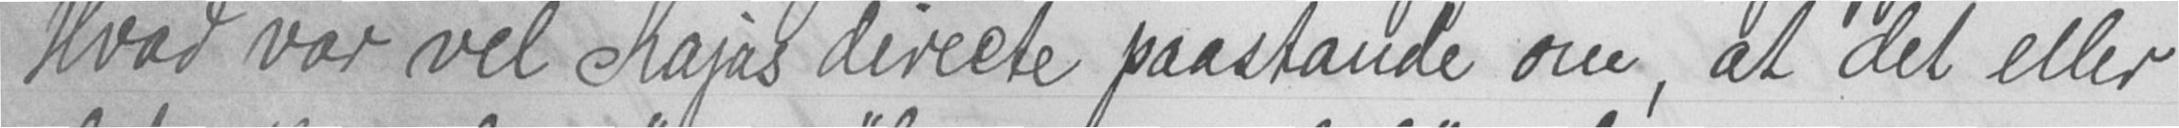Hvad var vel Kajas directe paastande om, at det eller
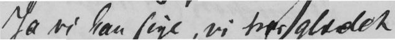Ja vi kan sige, vi har glædet
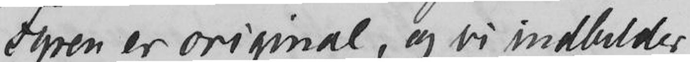Fyren er original, og vi indbilder
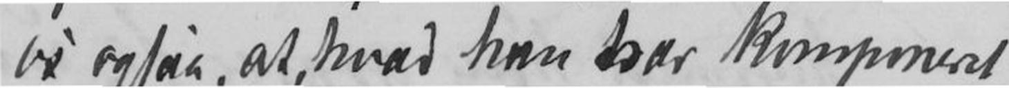os ogsaa, at, hvad han har komponeret
